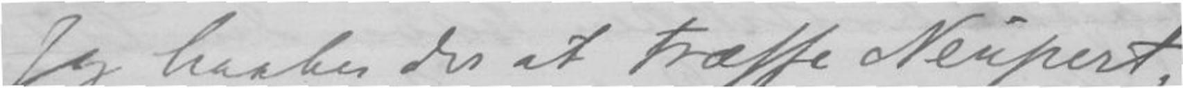Jeg haaber der at træffe Neupert,
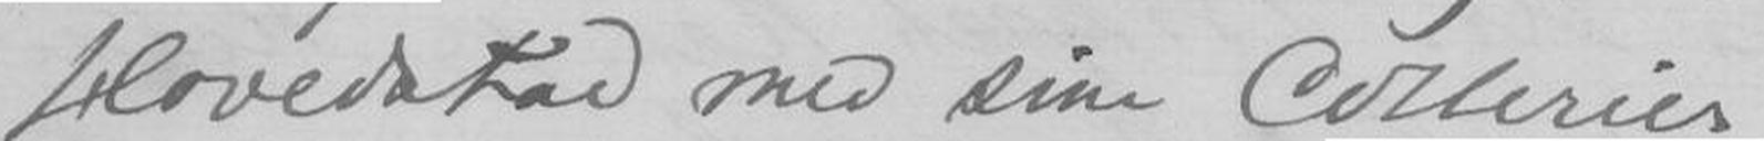Hovedstad med sine Cotterier.
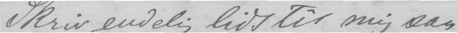Skriv endelig lidt til mig saa
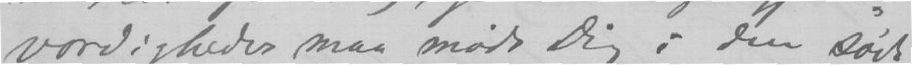vordighedes maa mødt Dig i den søde
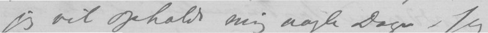jeg vil opholde mig nogle Dage. Jeg
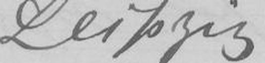Leipzig
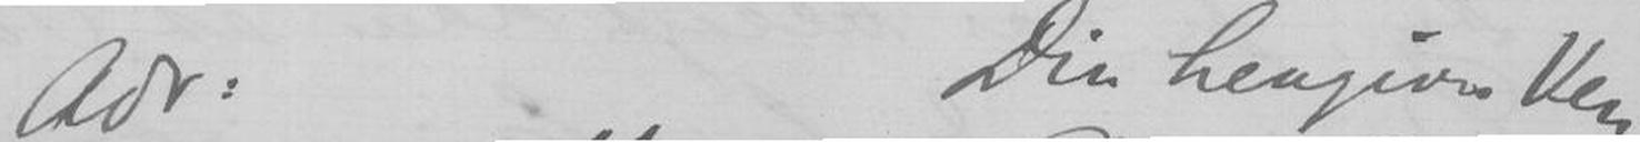Adr: Din hengivne Ven
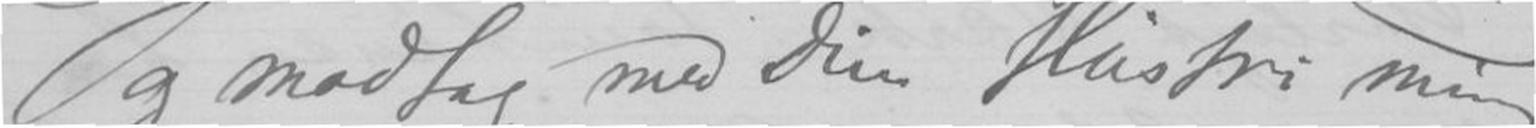og modtag med Din Hustru mine
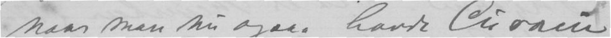naar man nu ogsaa havde Curans
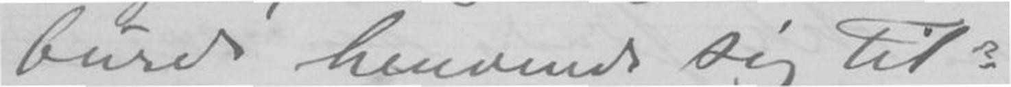burde henvende sig til?
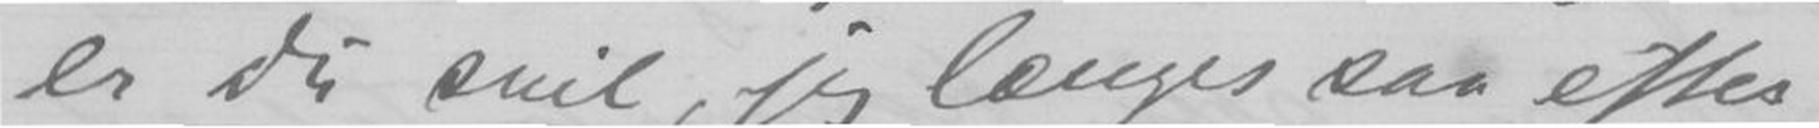er Du snil, jeg længes saa efter
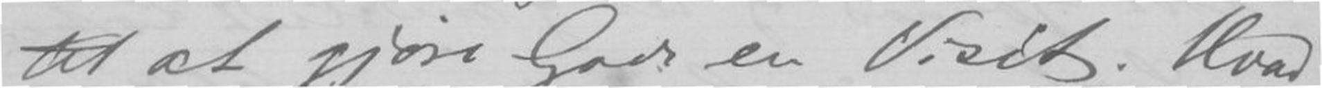til at gjøre Gade en Visit. Hvad
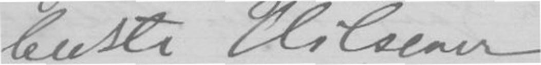bedste Hilsener
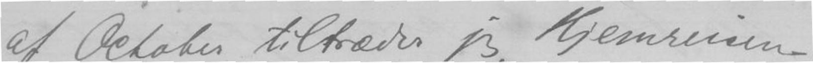af October tiltrædes jeg Hjemreisen
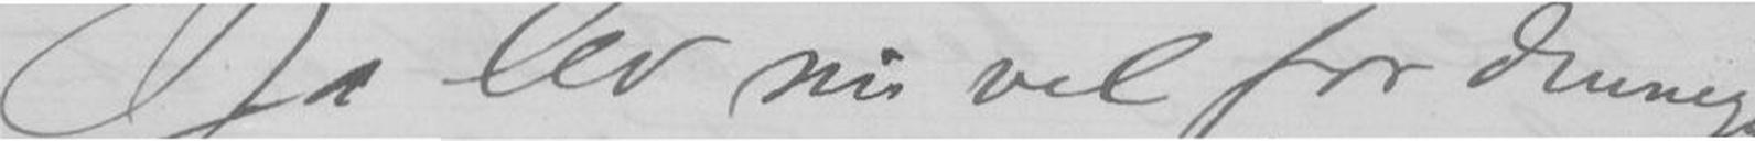Ja lev nu vel for dennegang
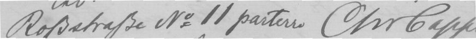Rofsstrasse No 11 parterre Chr. Capp
